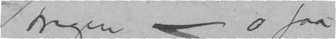Stregen < o saa
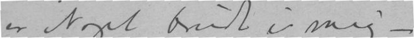er Noget brudt i mig -
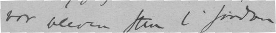var bleven sten i saadan
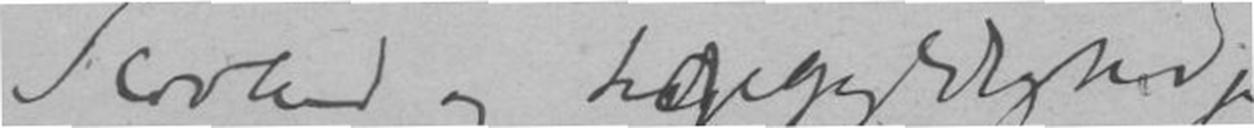Sløvhed og ligegyldighed jeg
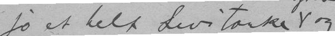jo et helt Livstanke og
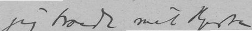jeg troede mit Hjerte
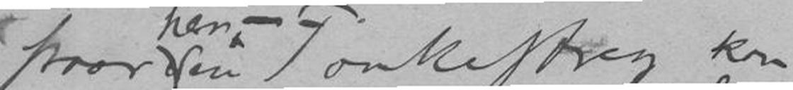staar en Tankestreg kan
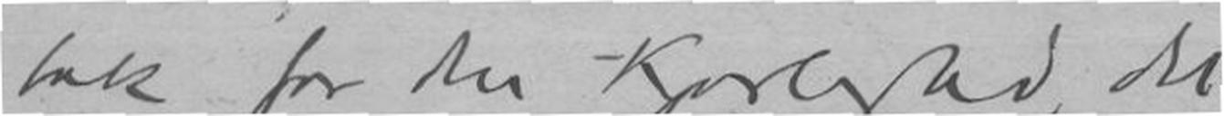tak for den Kjærlighed, det
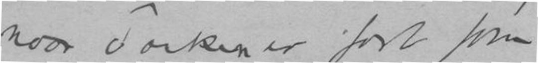naar Tanken er fast som
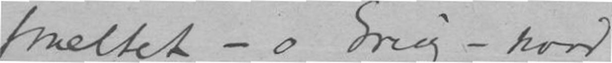smeltet - o Grieg - hvad
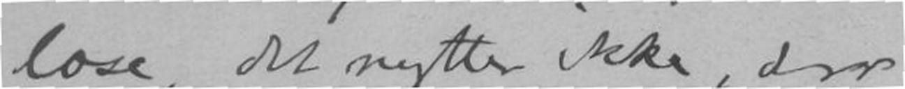løse. det nytter ikke, der
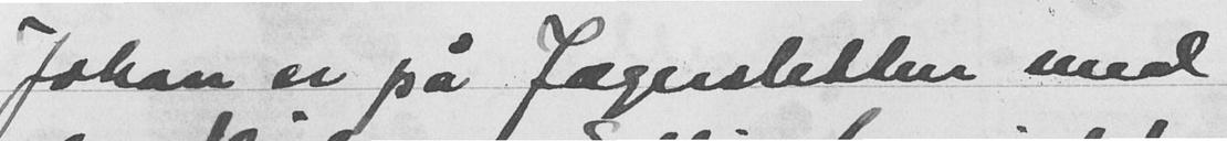Johan er på Fagersletten med
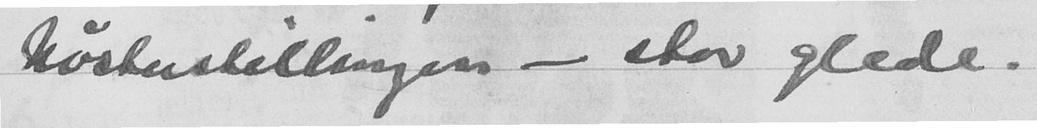høstutstillingen - stor glede.
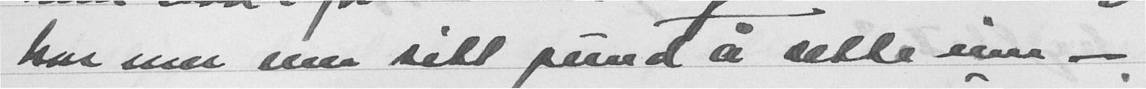har mer enn sitt pund å sette inn -
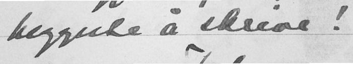begynte å skrive.
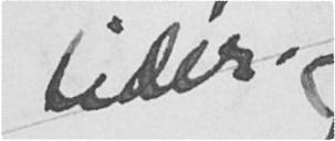tider.
